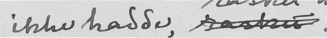ikke hadde, rasket
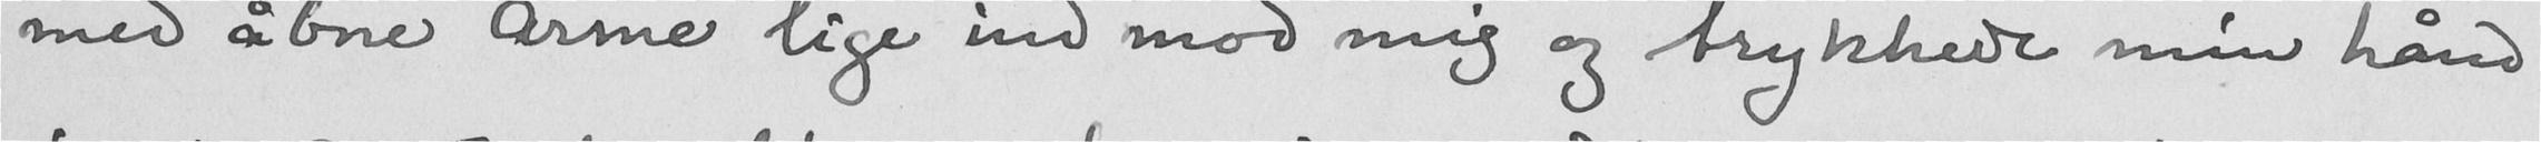med åbne arme lige ind mod mig og trykkede min hånd
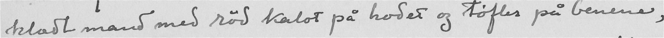klædt mand med rød kalot på hodet og tøfler på benene,
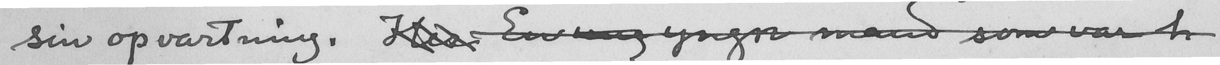sin opvartning. Her En ung yngre mand som var h
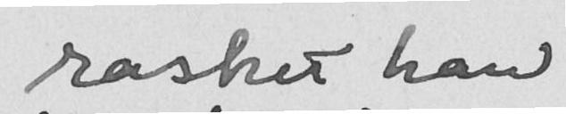rasket han
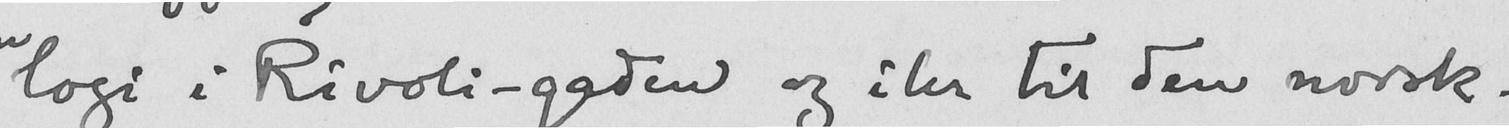logi i Rivoli-gaden og iler til den norsk-
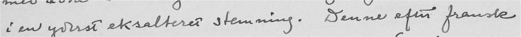i en yderst eksalteret stemning. Denne efter fransk
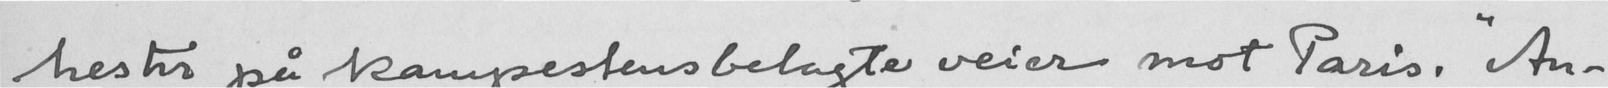hester på kampestensbelagte veier mot Paris. "An-
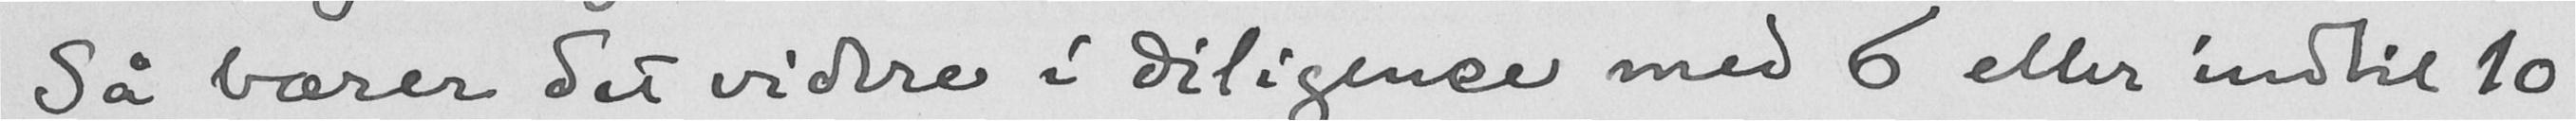Så bærer det videre i diligense med 6 eller indtil 10
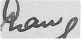han
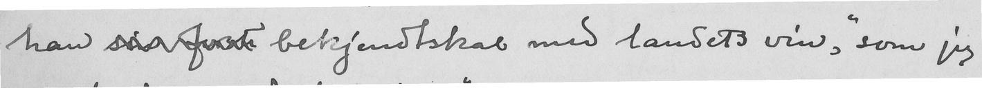han sin første bekjendtskab med landets vin, "som jeg
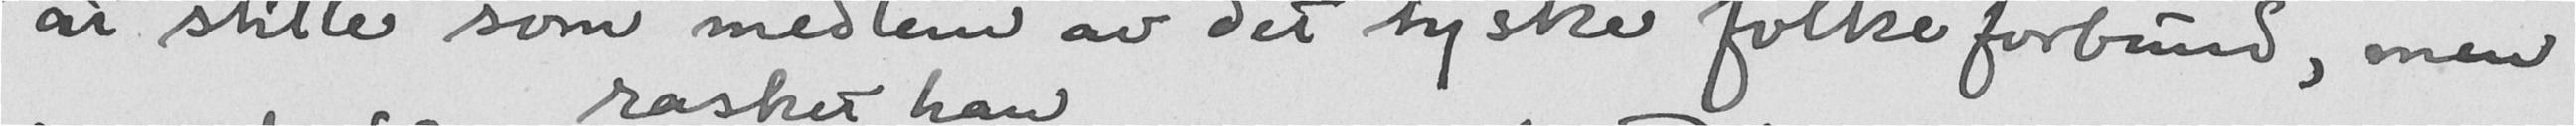at stille som medlem av det tyske folkeforbund, men
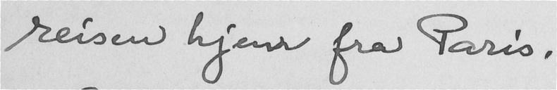reisen hjem fra Paris.
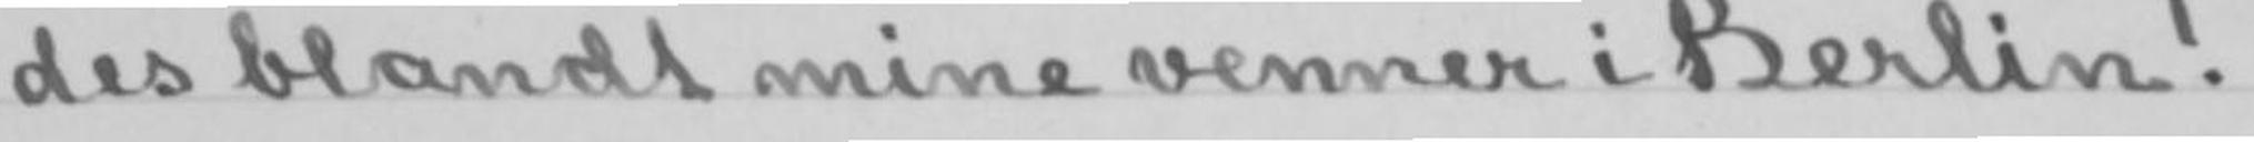des blandt mine venner i Berlin!
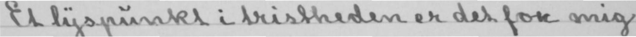Et lyspunkt i tristheden er det for mig
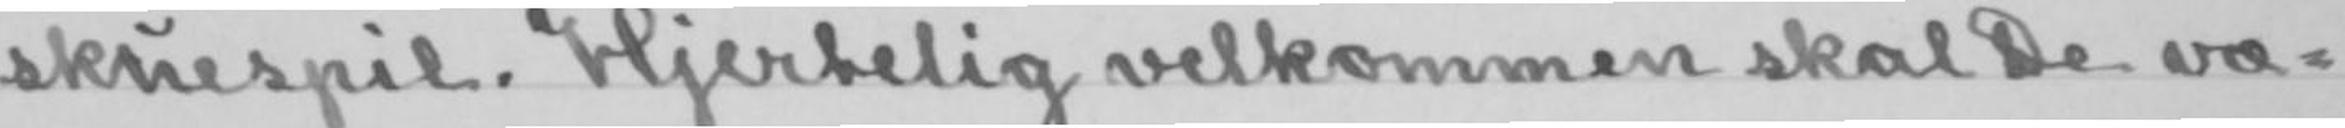skuespil. Hjertelig velkommen skal De væ-
